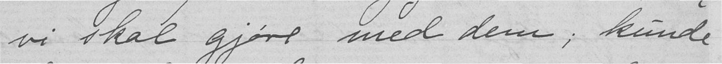vi skal gjøre med dem; kunde
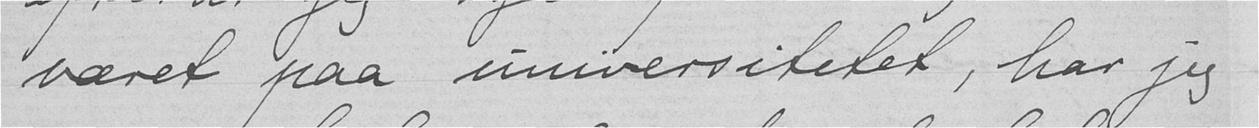været paa universitetet, har jeg
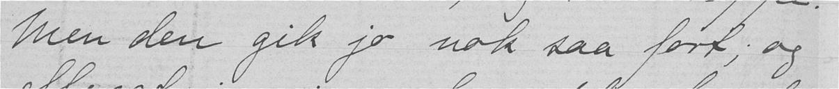Men den gik jo nok saa fort; og
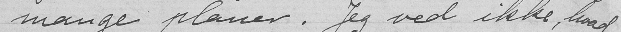mange planer. Jeg ved ikke, hvad
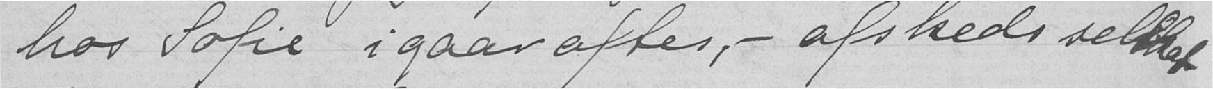hos Sofie igaar aftes, - afskedsselskab
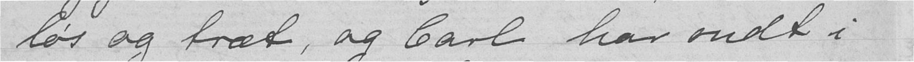løs og træt, og Carl har ondt i
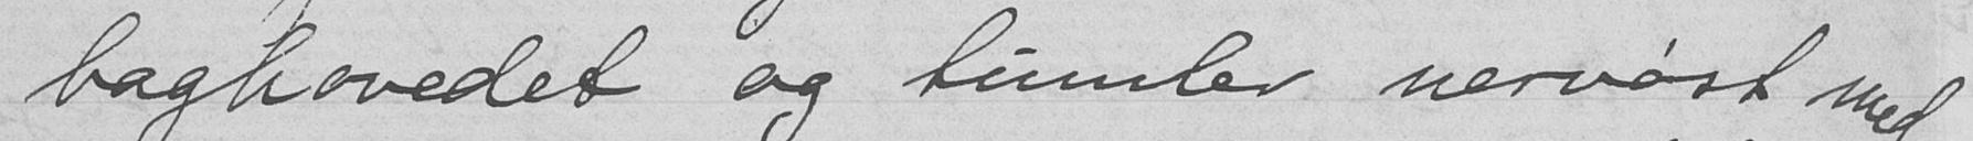baghovedet og tumler nervøst med
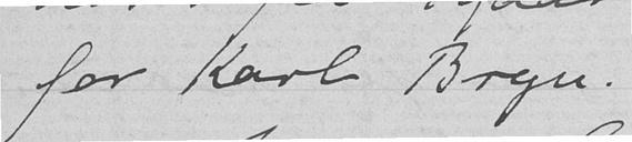for Karl Bryn.
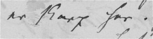er skarp her.
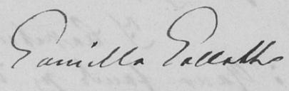Camilla Collett
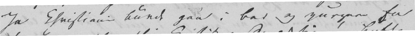Ja Christiania burde gaa i Bad og purgere for
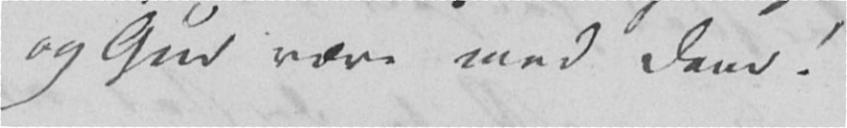og Gud være med Dem!
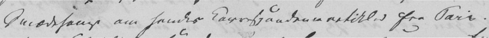Smædesange om hendes Korrespondenceartikler fra Paris.
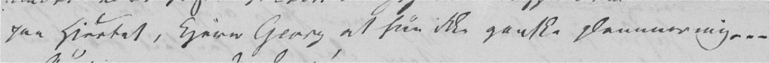paa Hjertet, kjere Georg at hun ikke ganske glemmer mig – – –
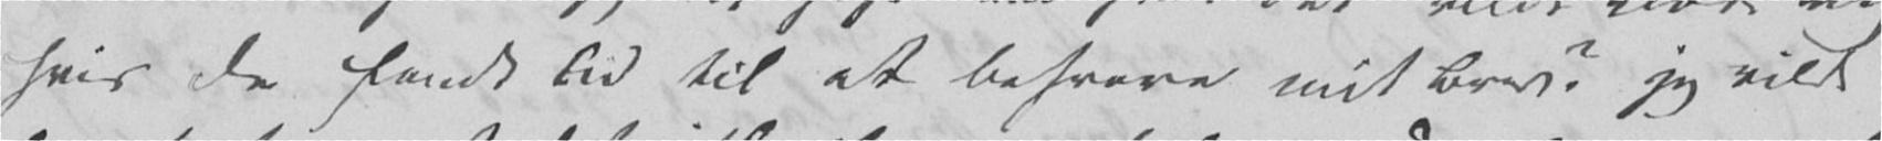hvis De fandt Tid til at besvare mit Brev? jeg vilde
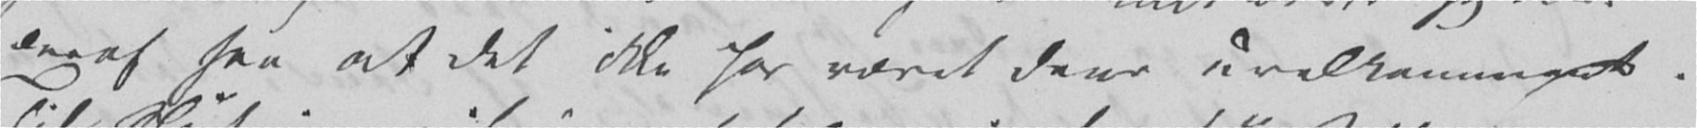deraf see at det ikke har været Dem uvelkomment.
